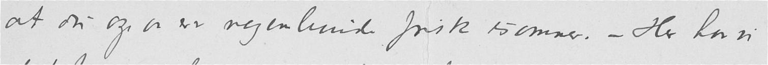at du ogsaa er nogenlunde frisk isommer. – Her har vi
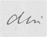din
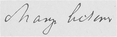Mange hilsener
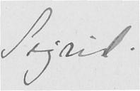Sigrid.
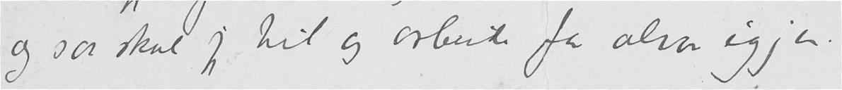og saa skal jeg til og arbeide for alvor igjen.
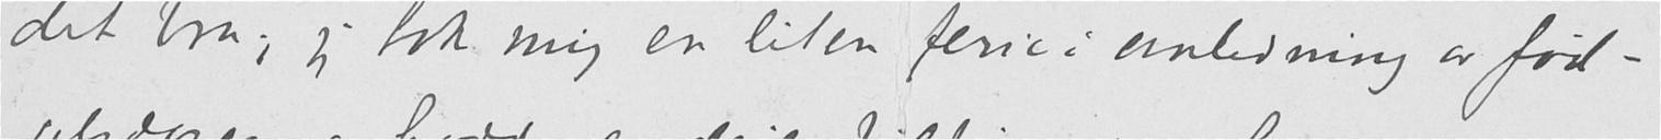det bra; jeg tok mig en liten ferie i anledning av fød-
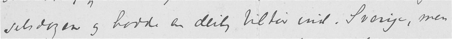selsdagen og hadde en deilig biltur ind i Sverige, men
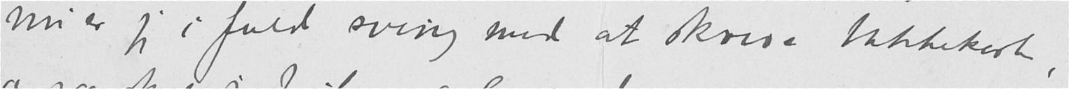nu er jeg i fuld sving med at skrive takkekort,
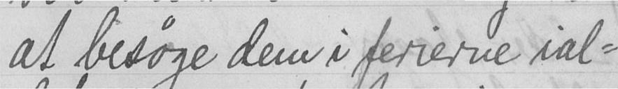at besøge dem i ferierne ial-
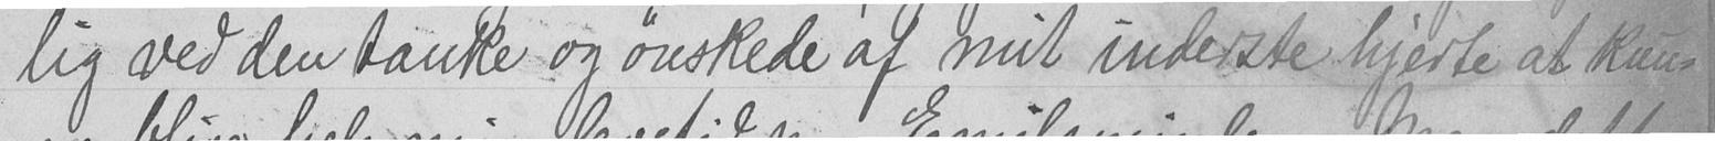lig ved den tanke og ønskede af mit inderste hjerte at kun-
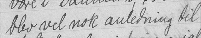blev vel nok anledning til
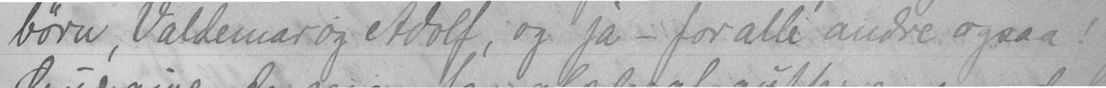børn, Valdemar og Adolf, og ja - for alle andre ogsaa!
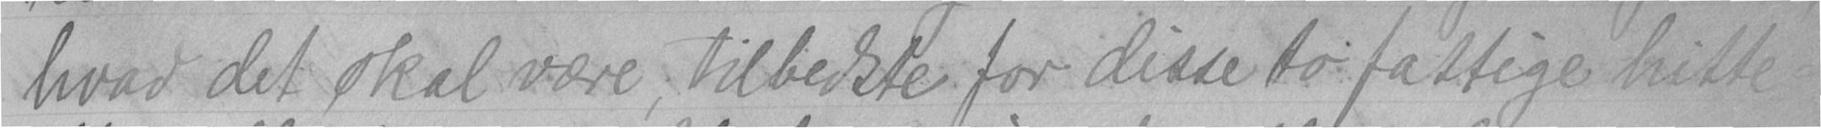hvad det skal være, tilbedste for disse to fattige hitte-
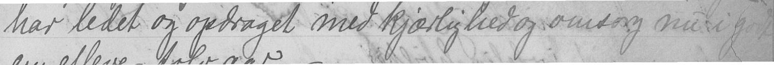har ledet og opdraget med kjærlighed og omsorg nu i godt
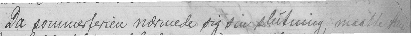Da sommerferien nærmede sig sin slutning, maatte tan-
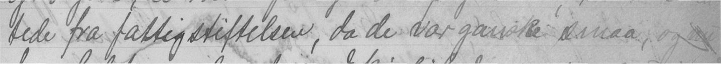tede fra fattigstiftelsen, da de var ganske smaa, og m
